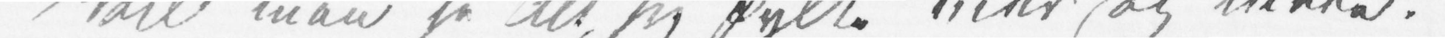Vil man se Alt sig fylke mer og mere.
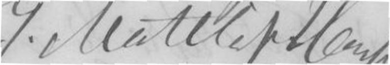G. Matthison-Hansen
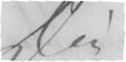Din
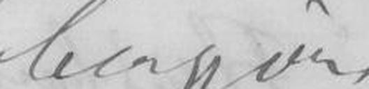hengivne
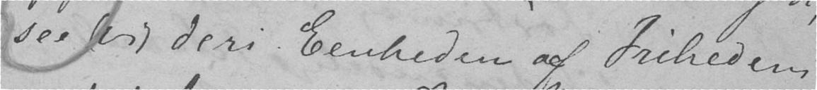see vi deri Eenheden af Frihedens
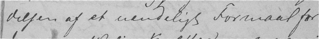delsen af et uendeligt Formaal for
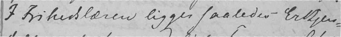I Frihedslæren ligger saaledes Erkjen-
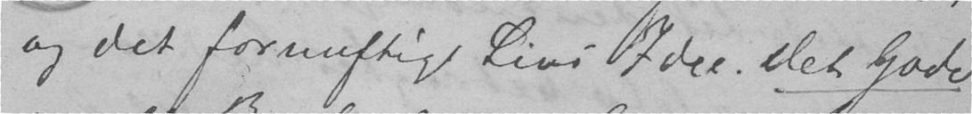og det fornuftige Livs Idee. Det Gode
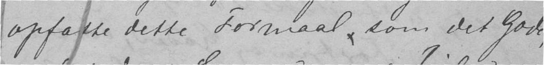opfatte dette Formaal, som det Gode,
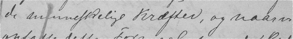de menneskelige Kræfter, og naar vi
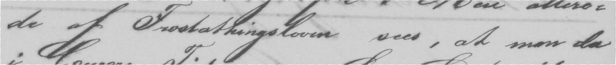de af Frostatningsloven sees, at man da
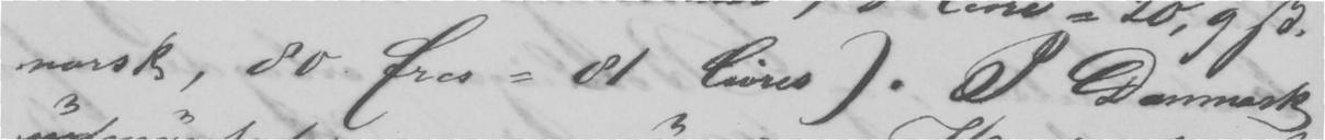norsk, 80 frcs = 81 livres). I Danmark
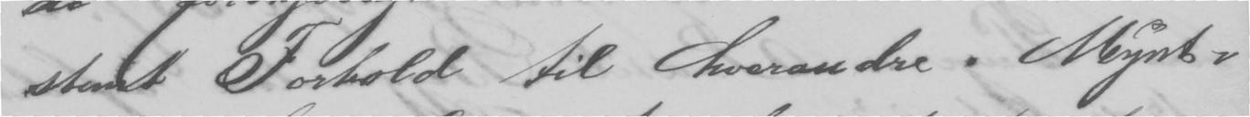stemt Forhold til hverandre . Mynt-
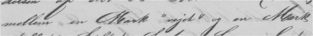mellem en Mark "vejet" og en Mark
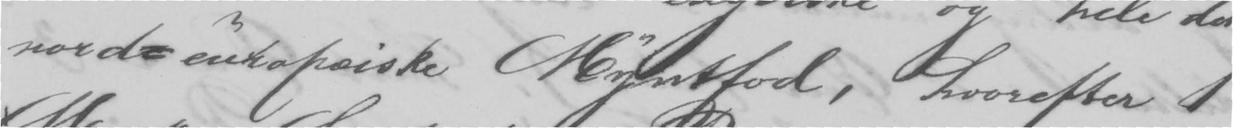nord-europeiske Myntfod, hvorefter 1
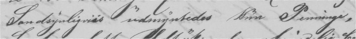Sandsynligvis udmyntedes kun Penninge,
